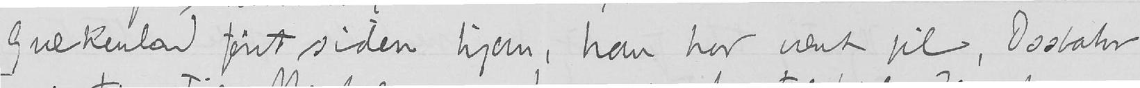Grekenland først siden hjem, han har vært jil, Ossbahr
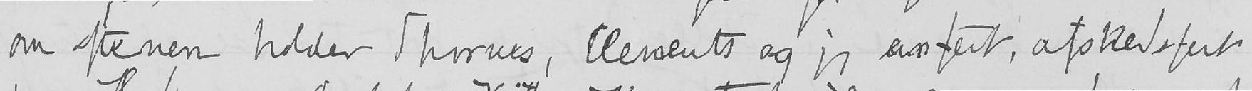om aftenen holder Thornes, Clements, og jeg en fest, afskedsfest
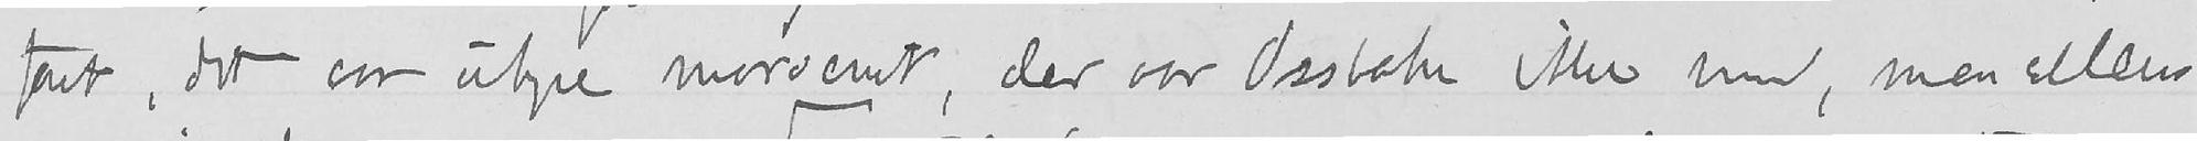fart, det var uhyre morsomt, der var Ossbahr ikke med, men ellers
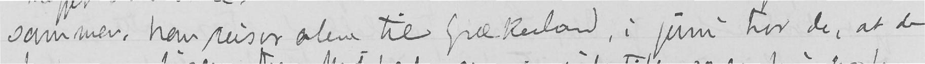sammen, han reiser alene til Grekenland, i juni tror de, at de
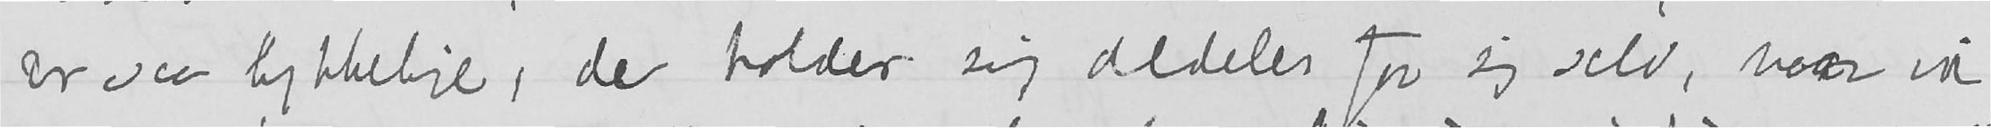er saa lykkelige, de holder sig aldeles for sig selv, naar vi
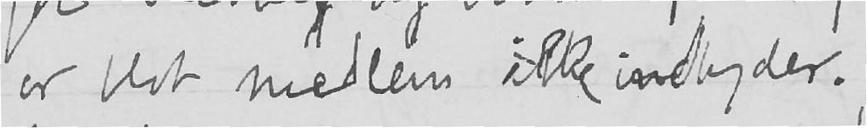er blot medlem ikke indbyder.
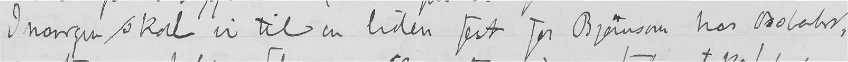Imorgen skal vi til en liden fest for Bjørnson hos Ossbahr,
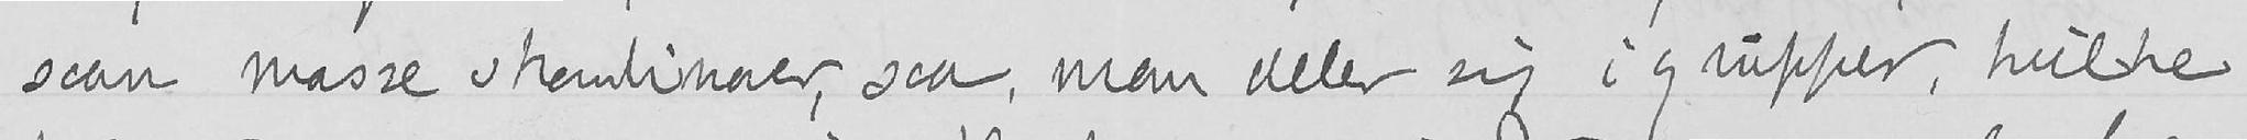saan masse skandinaver, saa, man deler sig i grupper, hvilke
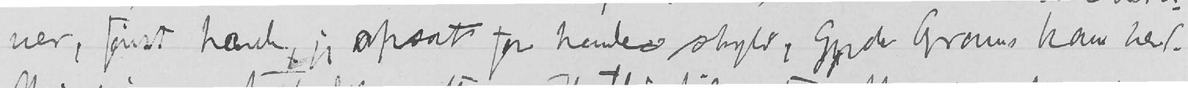ner, først havde jeg opsat for hendes skyld, Gyda Gram kom her.
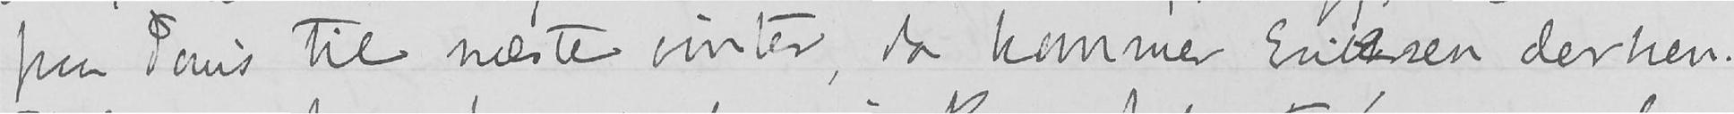paa Paris til næste vinter, da kommer Eriksen derhen.
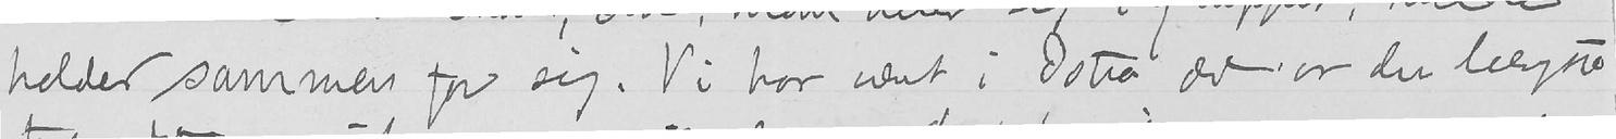holder sammen for sig. Vi har vært i Ostia det er den længste
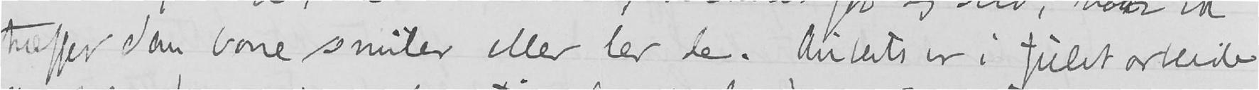træffer dem bare smiler eller ler de. Auberts er i fuldt arbeide
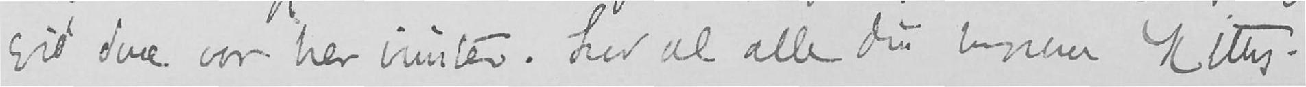gid dere var her ivinter. Lev vel alle din hengivne Kitty.
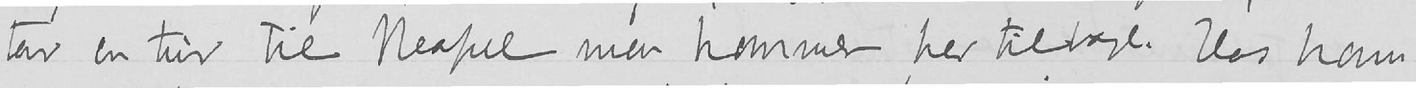tar en tur til Neapel men kommer her tilbage. Hos ham
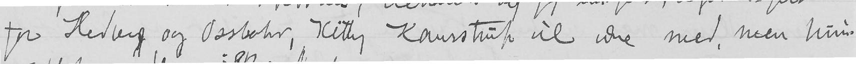for Hedberg og Ossbahr, Kitty Kamstrup vil være med, men hun
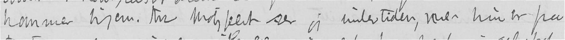kommer hjem. Fru Motzfeldt ser jeg undertiden, men hun er paa
